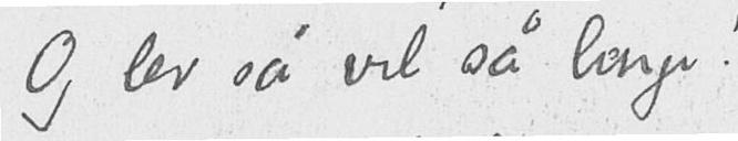Og lev så vel så længe.
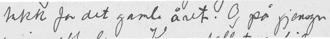takk for det gamle året. Og på gjensyn
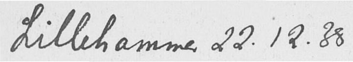Lillehammer 22.12.38
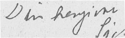Din hengivne
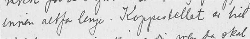innen altfor lenge. Koppestellet er til
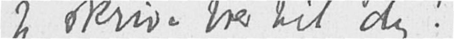jeg skrive brev til dig!
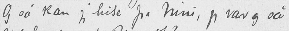Og så kan jeg hilse fra Nini, jeg var og så
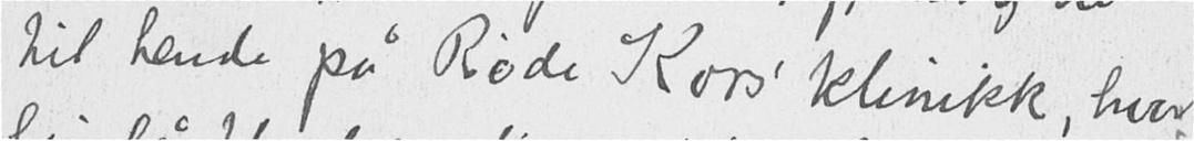til hende på Røde Kors klinikk, hvor
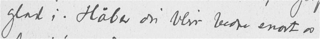glad i. Høber du blir bedre snart av
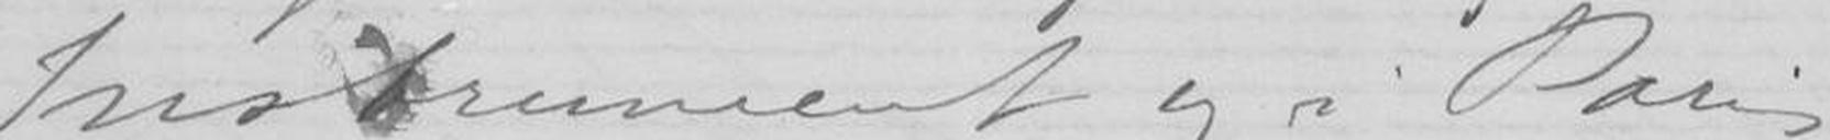Instrument og i Paris lader
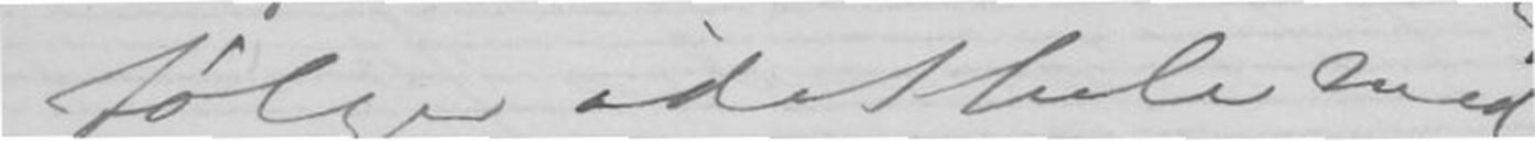og følger idethele med
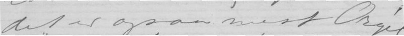det er ogsaa mest Orgel
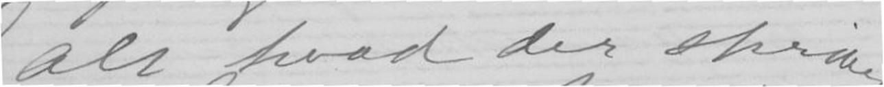Alt hvad der skrives
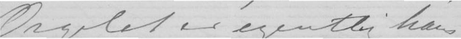Orgelet er egentlig hans
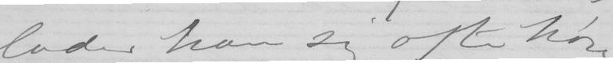han sig ofte høre,
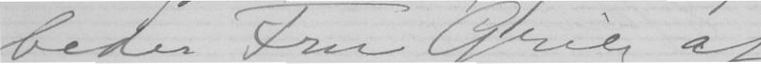bedes Fru Grieg at
